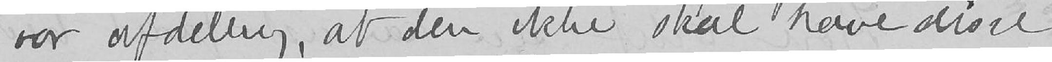vor afdeling, at den ikke skal have disse
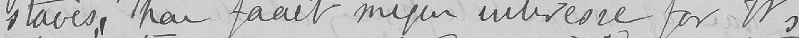staves, har faaet megen interesse for Ws
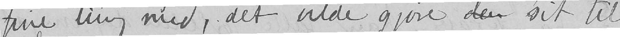fine ting med, det vilde gjøre den sit til
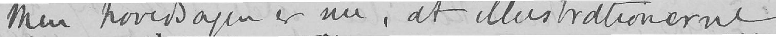Men hovedsagen er nu, at illustrationerne
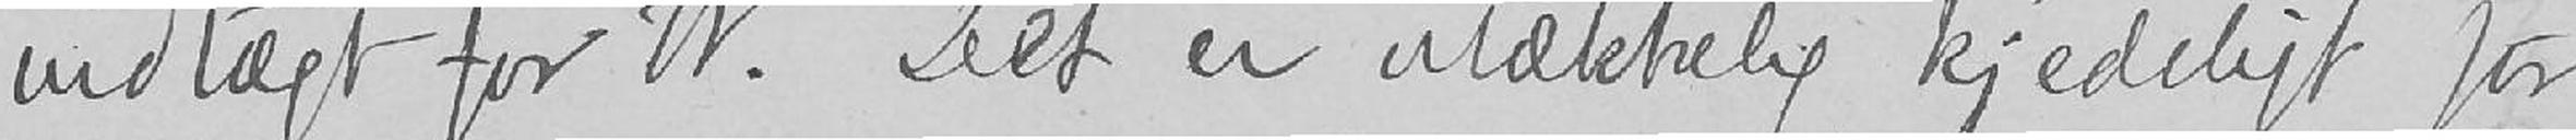indtægt for W. Det er utækkelig kjedeligt for
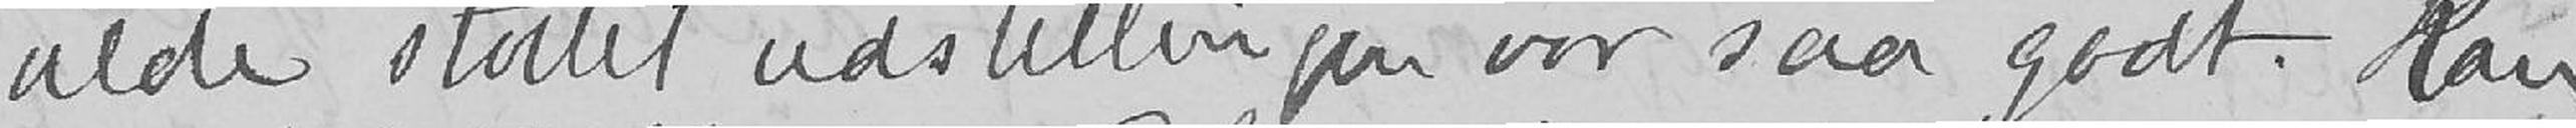vilde støttet udstillingen vor saa godt. Kan
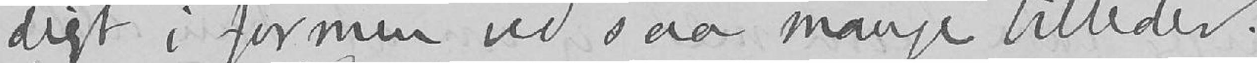digt i formen ved saa mange billeder.
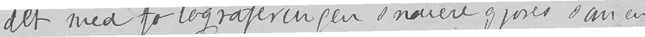det med fotograferingen snarere gjøres som en
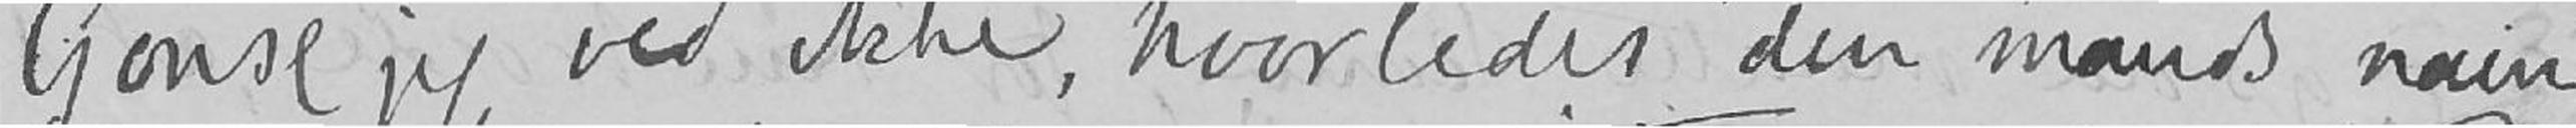Gonse jeg ved ikke, hvorledes den mands navn
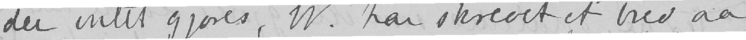der intet gjøres, W. har skrevet et brev, aa
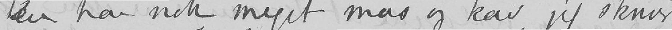Du har nok meget mas og kav, jeg skriver
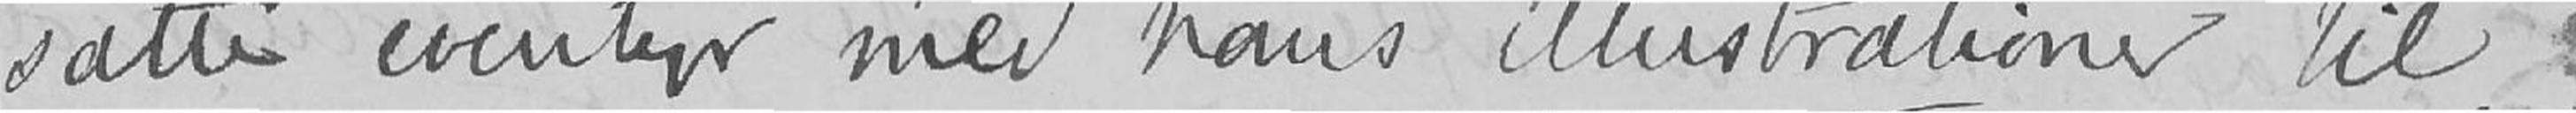satte eventyr med hans illustrationer til.
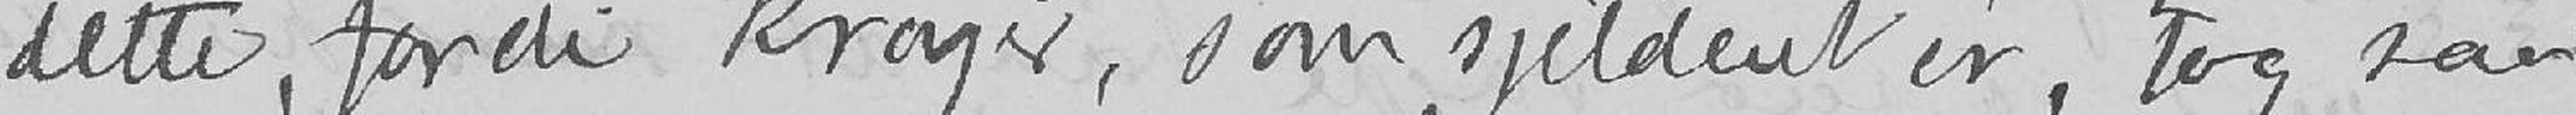dette, fordi Krøyer, som sjeldent er, tog saa-
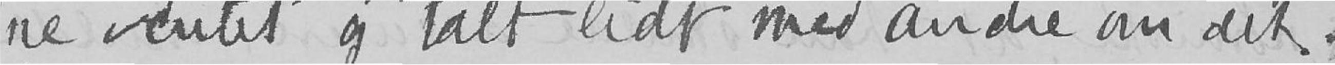ne ventet og talt lidt med andre om det.
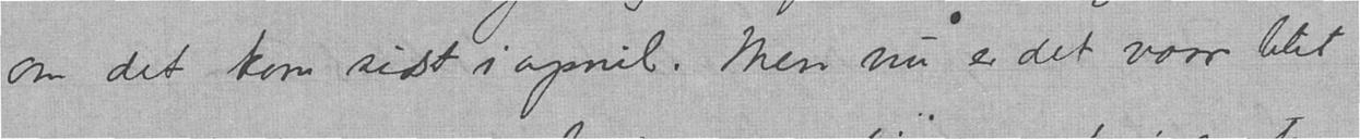om det kom sidst i april. Men nu er det vaar blit
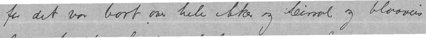for det var bart over hele Aker og leirsol og blaaveis
